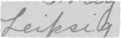Leipsig
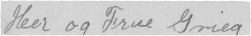Heer og Frue Grieg
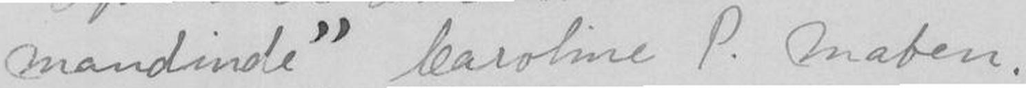mandinde " Caroline P. Maben.
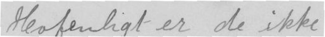Heofenligt er de ikke
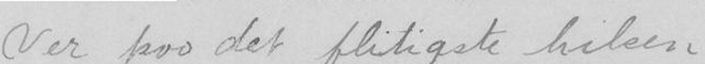Ver poo det flitigste hilsen,
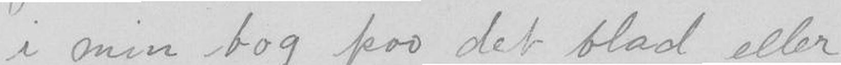i min bog poo det blad eller
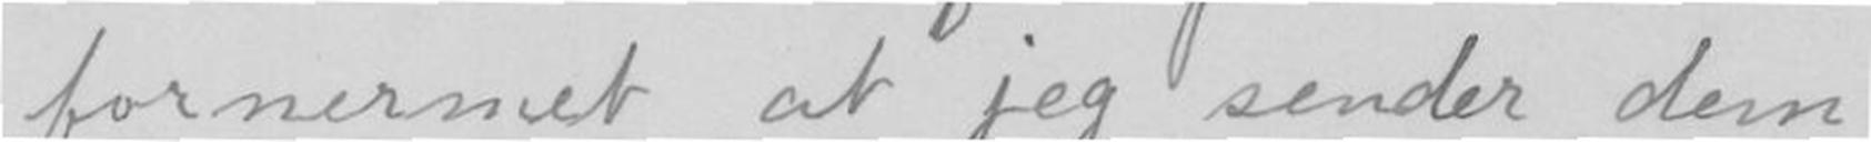fornermet at jeg sender dem
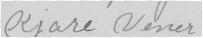Kjære Vener
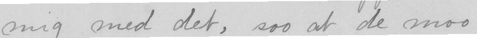mig med det, soo at de moo
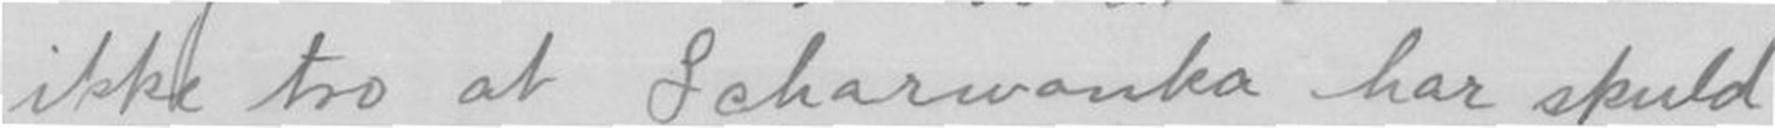ikke tro at Scharwanka har skuld
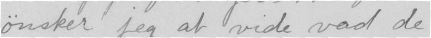ønsker jeg at vide vad de
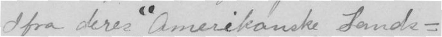I fra deres "Amerikanske Lands-
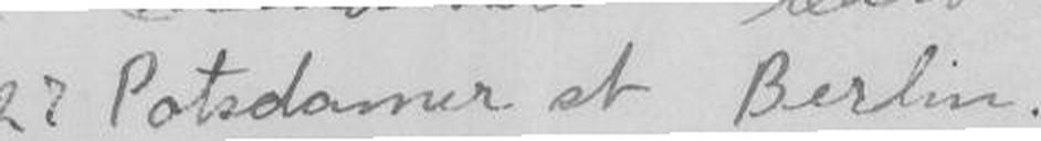27 Potsdamer st Berlin.
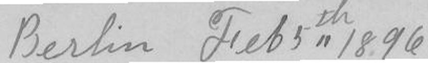Berlin Feb 5th 1896
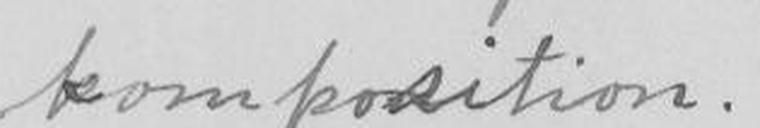komposition.
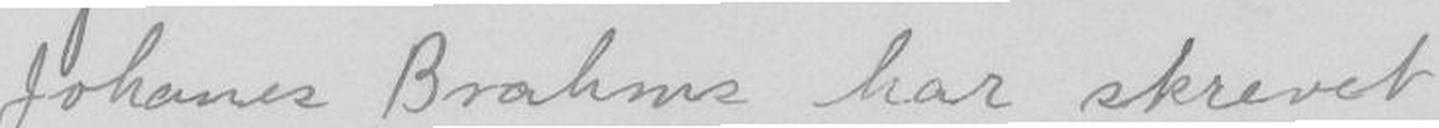Johanes Brahms har skrevet
